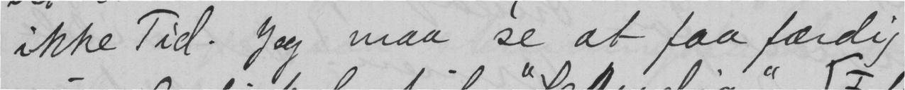ikke Tid. Jeg maa se at faa færdig
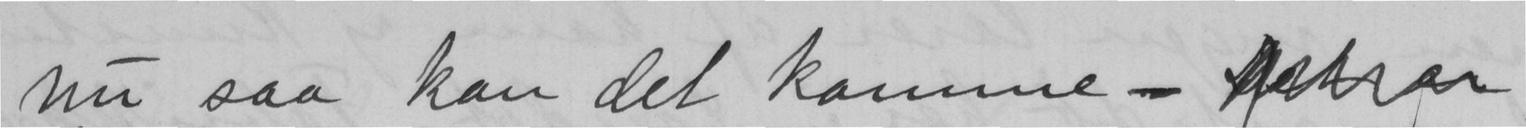nu saa kan det komme -
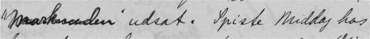"Maskeraden" udsat. Spiste Middag hos
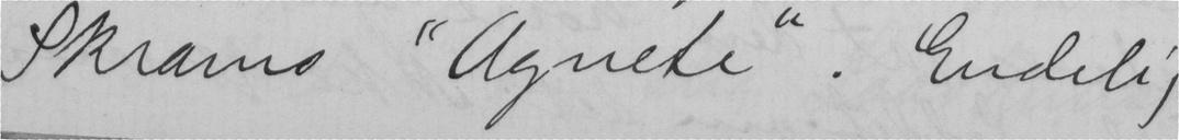Skrams "Agnete". Endelig
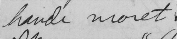havde moret
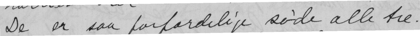De er saa forfærdelige søde alle tre.
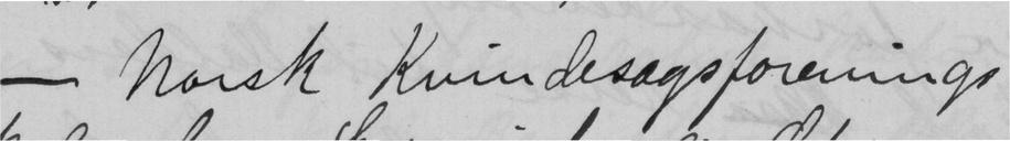- Norsk Kvindesagsforenings
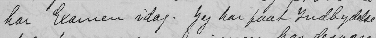har Examen idag. Jeg har faat Indbydelse
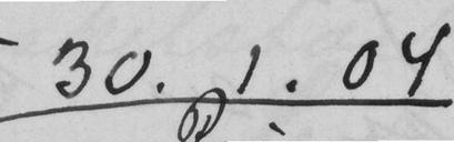30.1.04
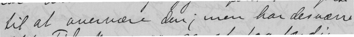til at overvære den; men har desværre
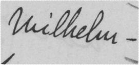Wilhelm-
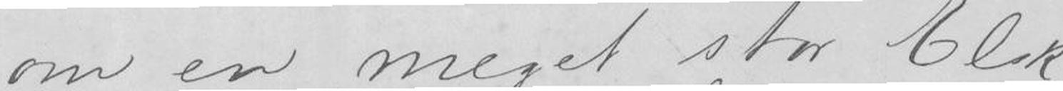om en meget stor Elsk-
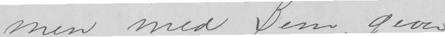men med Dem, giver
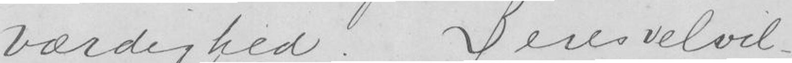værdighed. Deres velvil-
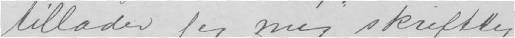tillader jeg mig skriftlig
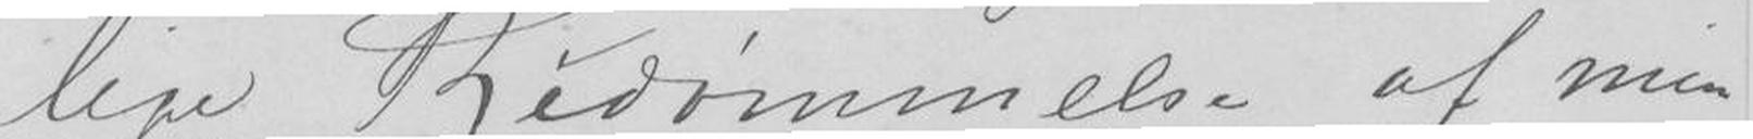lige Bedømmelse af min
